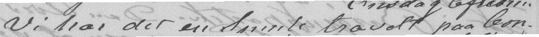Vi har det en Smule travelt pa Con-
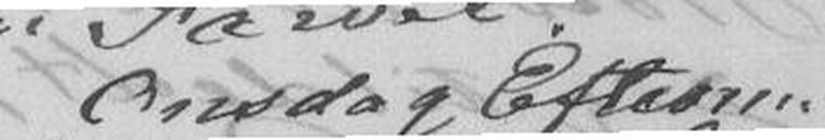Onsdag Efterm.
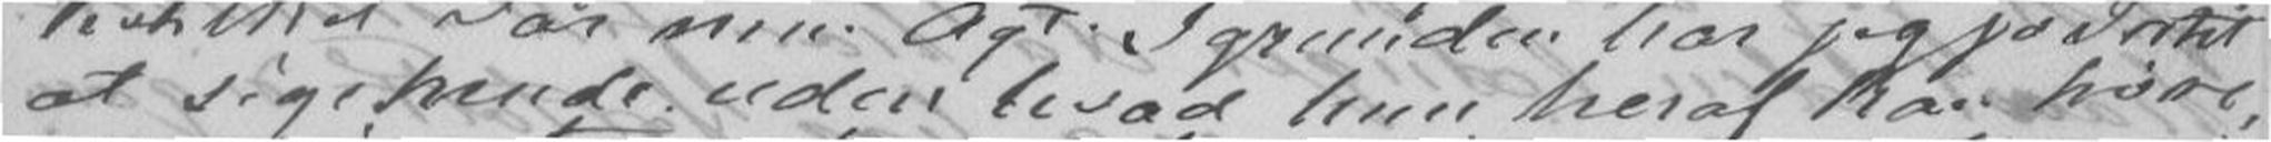at sige hende uden hvad hun heraf kan høre,
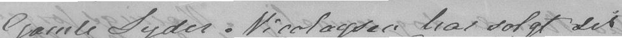Gamle Lyder Nicolaysen har solgt det
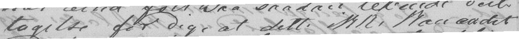tagelse for Dig, at dette ikke kan andet
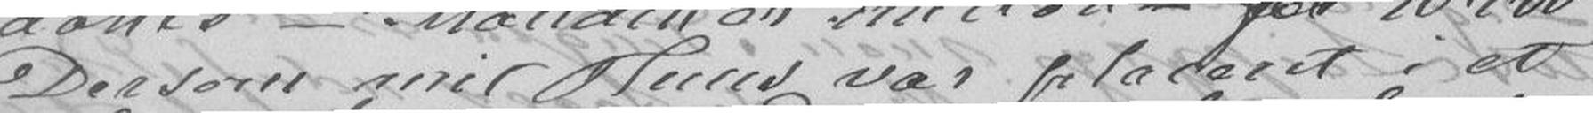Dersom mit Huus var plaseret i et
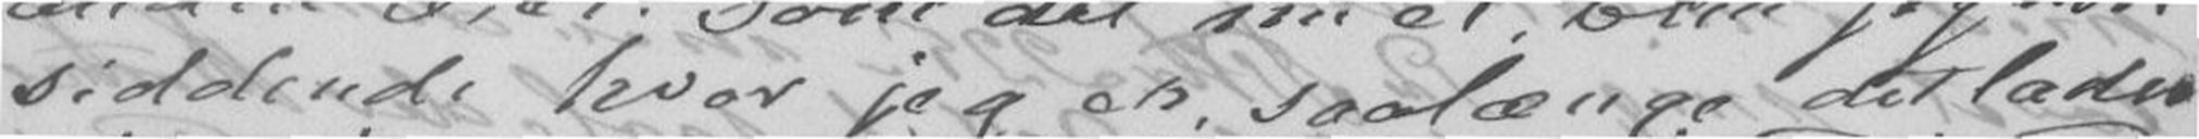siddende hvor jeg er saalænge det lader
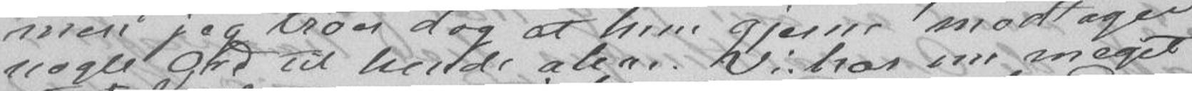nogle ord til hende alene. Vi har nu meget
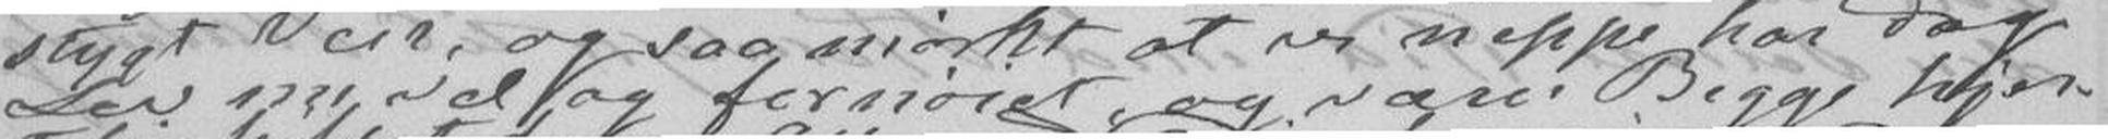Lev nu vel og fornøiet, og være Begge hjer-
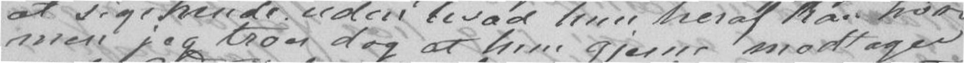men jeg troer dog at hun gjerne modtager
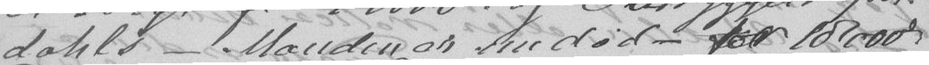dahls - Manden er nu død - for 18000do
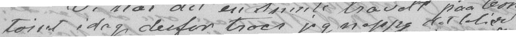toiret idag derfor troer jeg neppe det blive
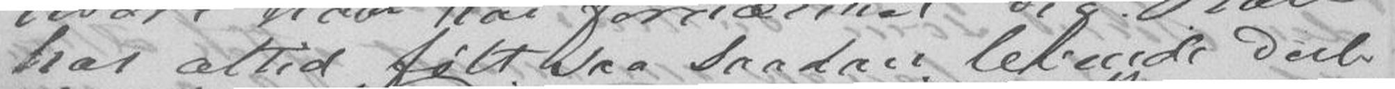har altid følt saa saadan levende Deel-
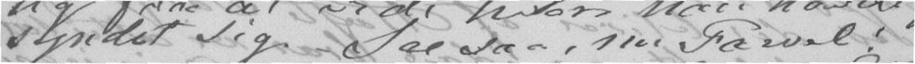syndet sig. - See saa, nu Farvel!
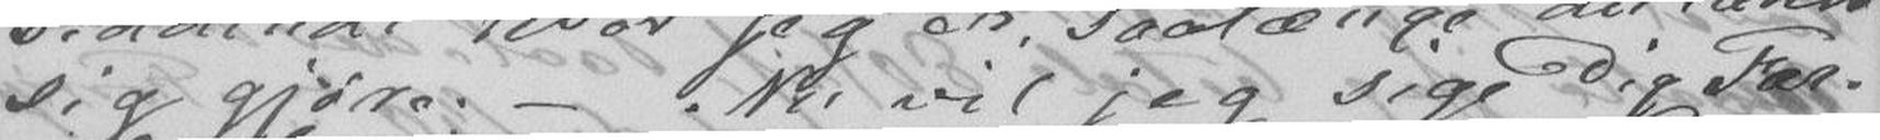sig gjøre. - Nu vil jeg sige Dig Far-
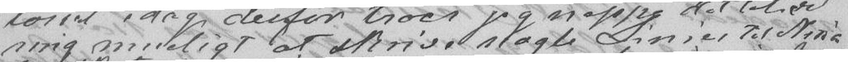mig mueligt at skrive nogle Linier til Nina
